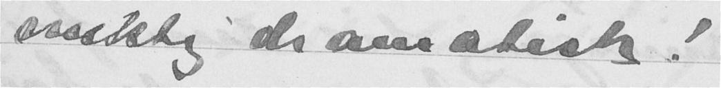mektig dramatisk!
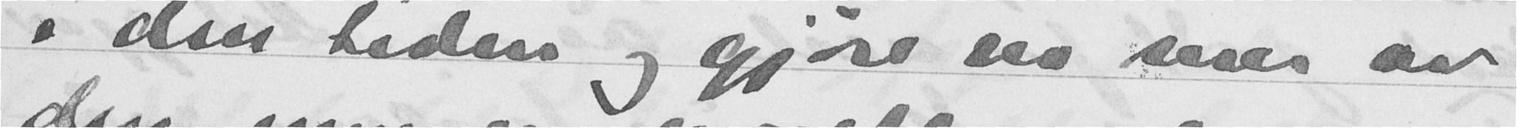i den tiden og gjøre no mer av
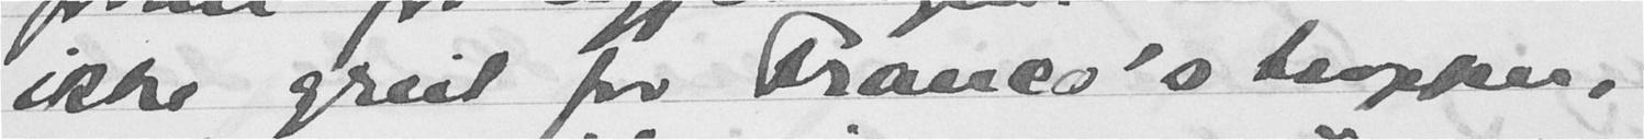ikke greit for Franco's tropper,
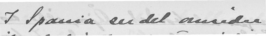I Spania ser det omsider
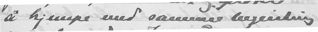å kjempe med samme begeistring
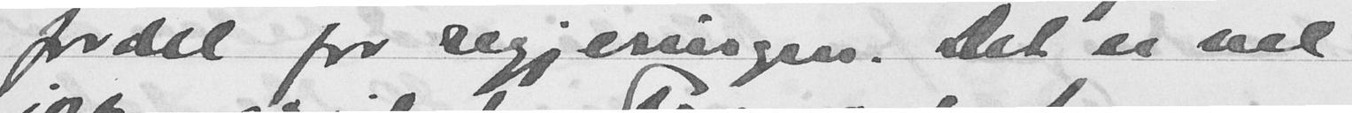fordel for regjeringen. Det er vel
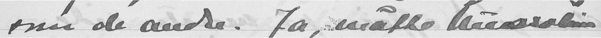som de andre. Ja, måtte Mussolini
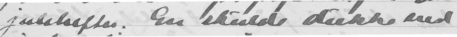julehefter. En skulde dukke ned
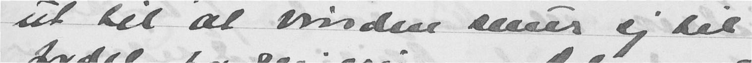ut til at vinden snur sig til
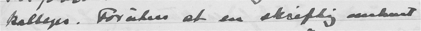kolleger. Foruten at en skriftlig omkost
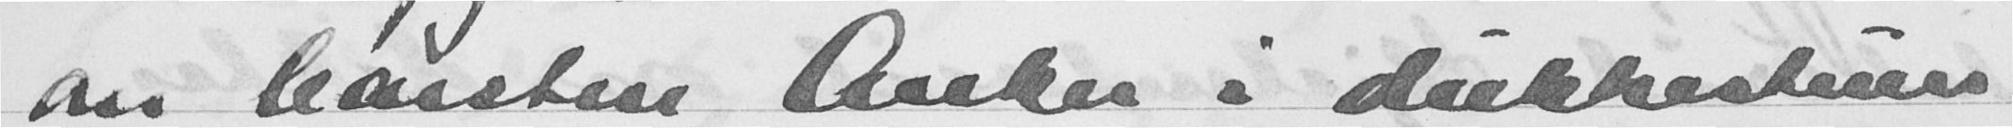Om Karsten Anker i dukkestuen
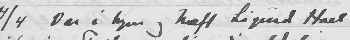4/4 Var i byen og traff Sigurd Hoel
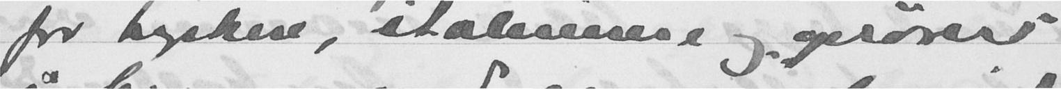for tyskere, italienere og oprørere
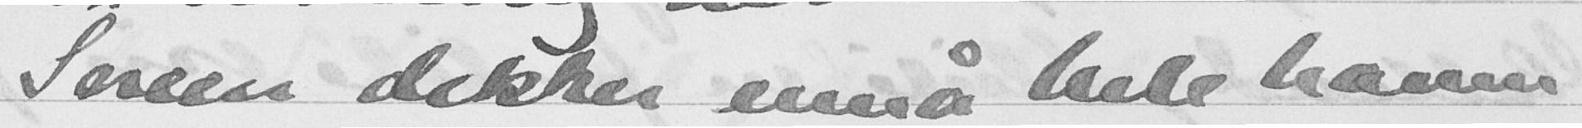Sneen dekker ennå hele haven
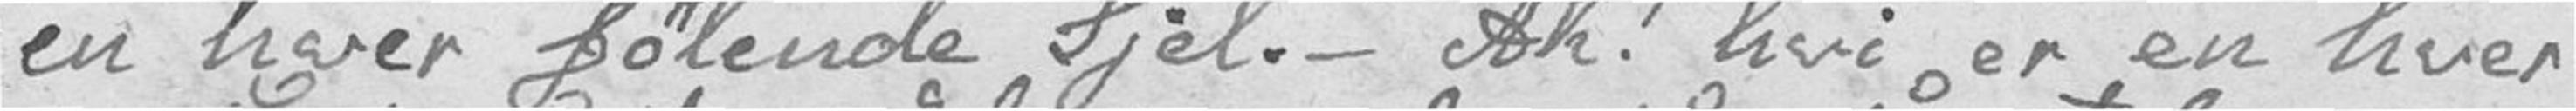en hver følende Sjel. – Ak! hvi er en hver
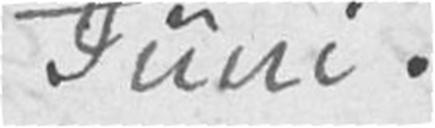Juni.
